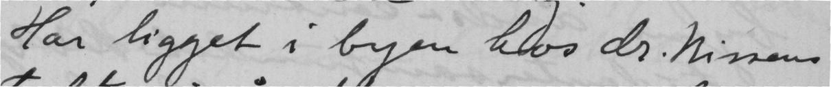Har ligget i byen hos dr Nissens
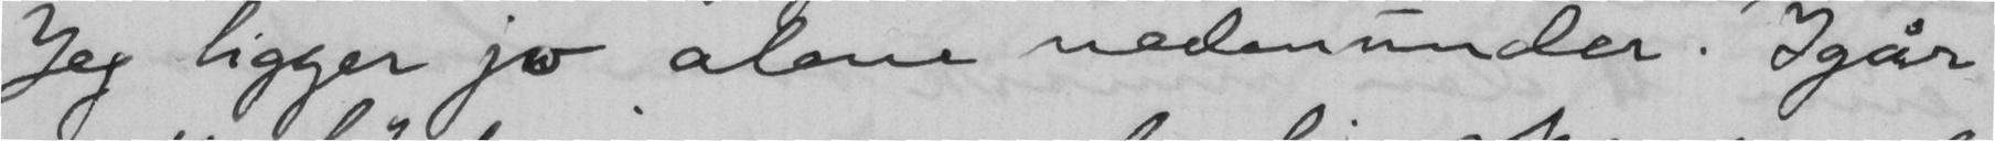Jeg ligger jo alene nedenunder. Igår
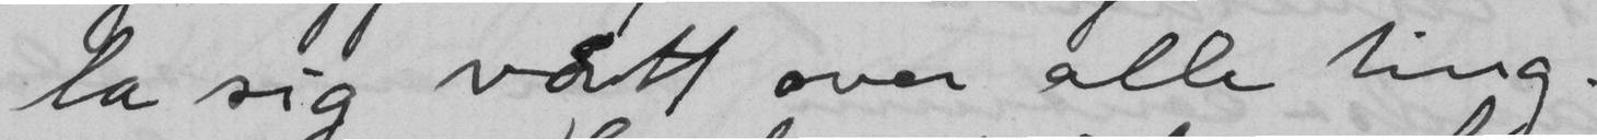la sig vått over alle ting.
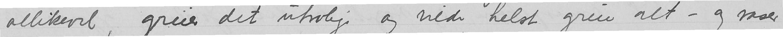allikevel, greier det utrolige og vilde helst greie alt – og raser
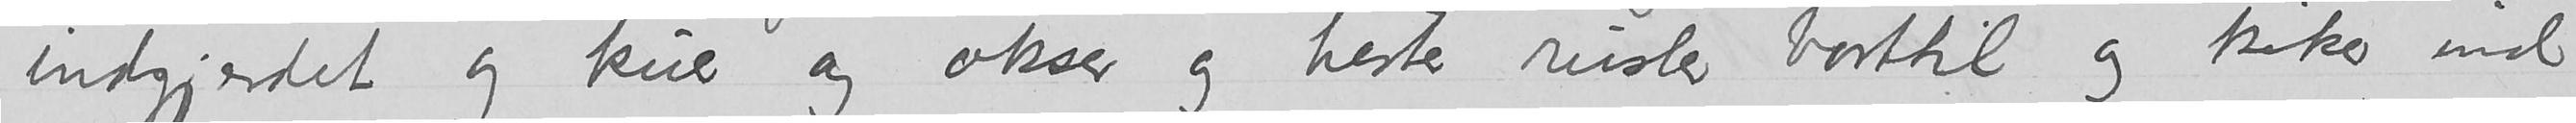indgjerdet og kuer og okser og hester rusler borttil og kiker ind
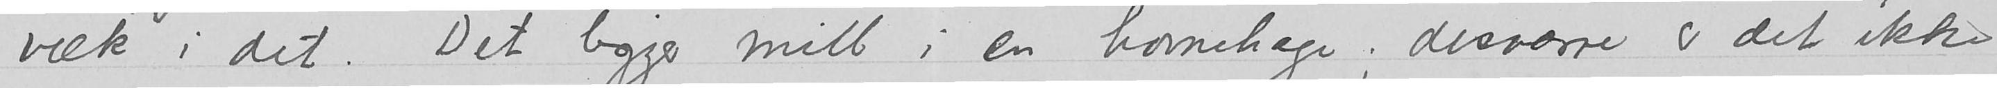væk i det. Det ligger mitt i en tornehage; dessværre er det ikke
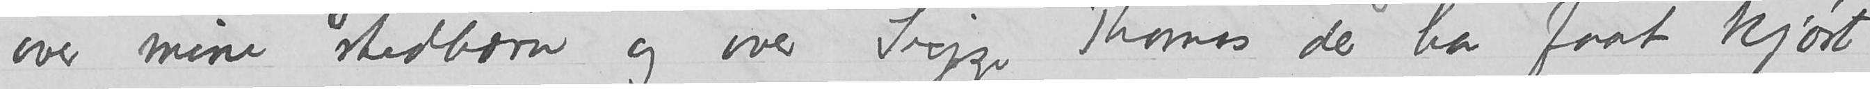over mine stedbørn og over Sigge Thomas der har faat kjørt
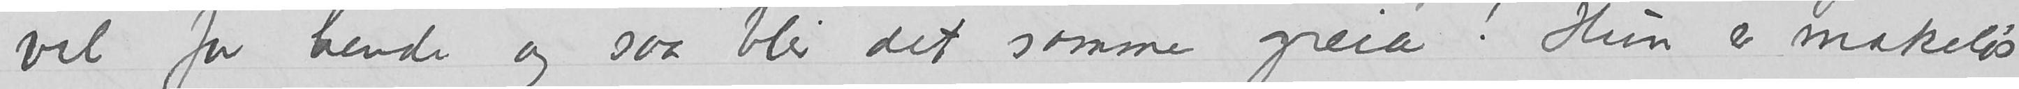vel for hende og saa blir det samme greia! Hun er makeløs
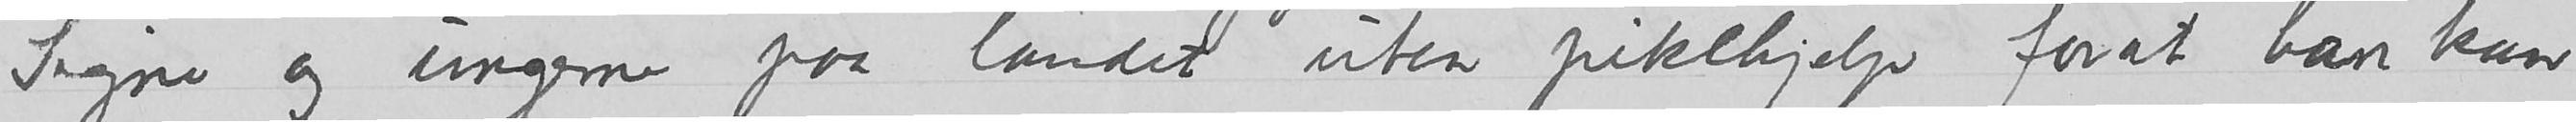Signe og ungerne paa landet uten pikehjelp for at han kan
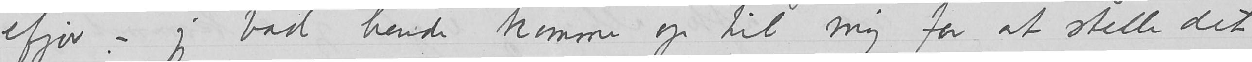ifjor – jeg bad hende komme op til mig for at stelle det
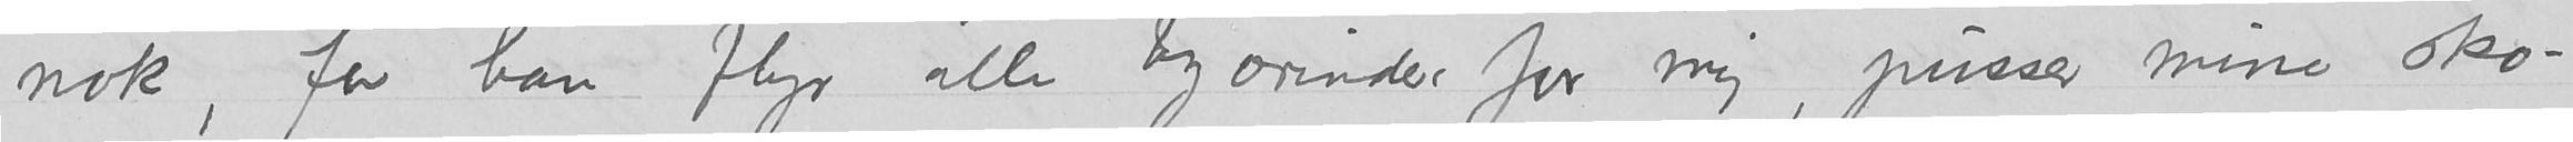nok, for han flyr alle byærinder for mig, pusser mine sko –
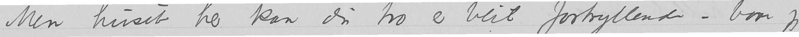Men huset her kan du tro er blit fortryllende – bare jeg
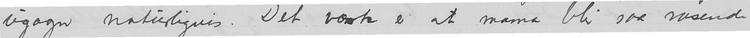ugagn naturligvis. Det værste er at mama blir saa rasende
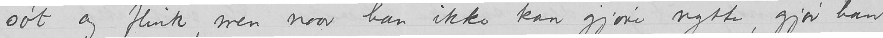søt og flink, men naar han ikke kan gjøre nytte, gjør han
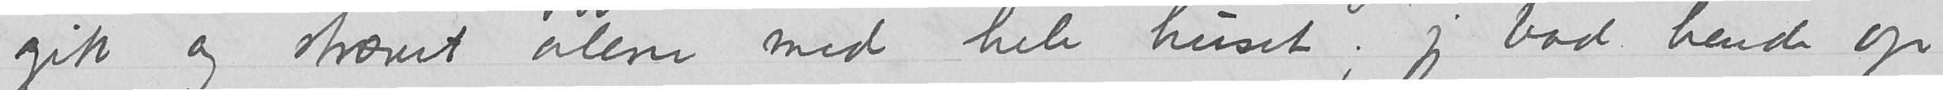gik og strævet alene med hele huset; jeg bad hende op
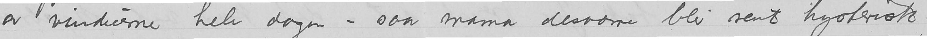av vinduerne hele dagen – saa mama desværre blir rent hysterisk;
